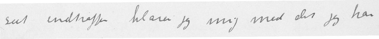seet indtræffer klarer jeg mig med det jeg har
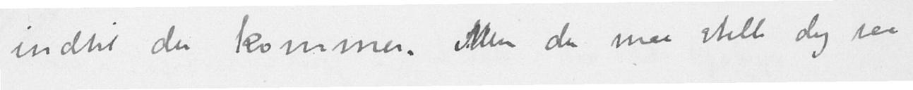indtil du kommer. Men du maa stille dig saa
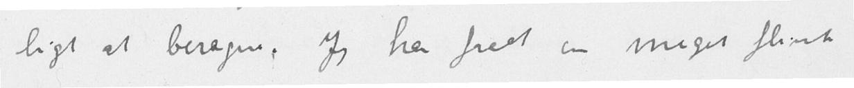ligt at berægne. Jeg har faaet en meget flink
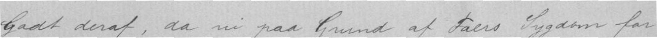Godt deraf, da vi paa Grund af Faers Sygdom for
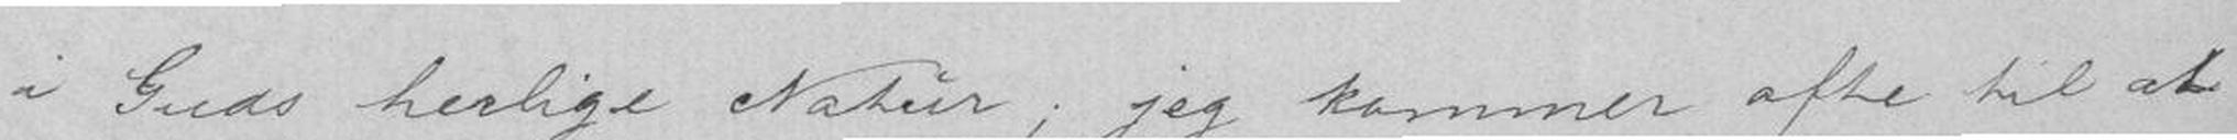i Guds herlige Natur; jeg kommer ofte til at
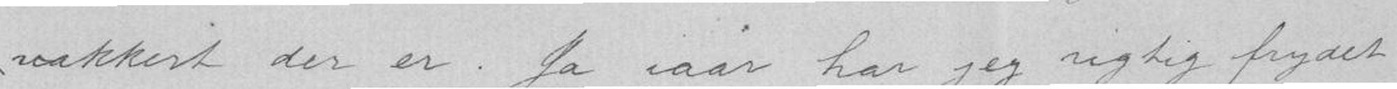vakkert der er. Ja iaar har jeg rigtig frydet
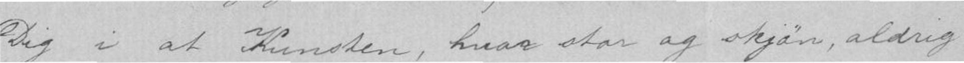Dig i at Kunsten, hvor stor og sjøn, aldrig
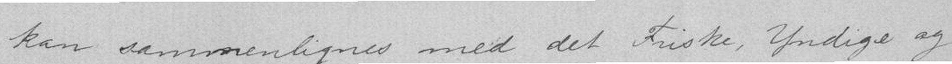kan sammenlignes med det Friake, Yndige og
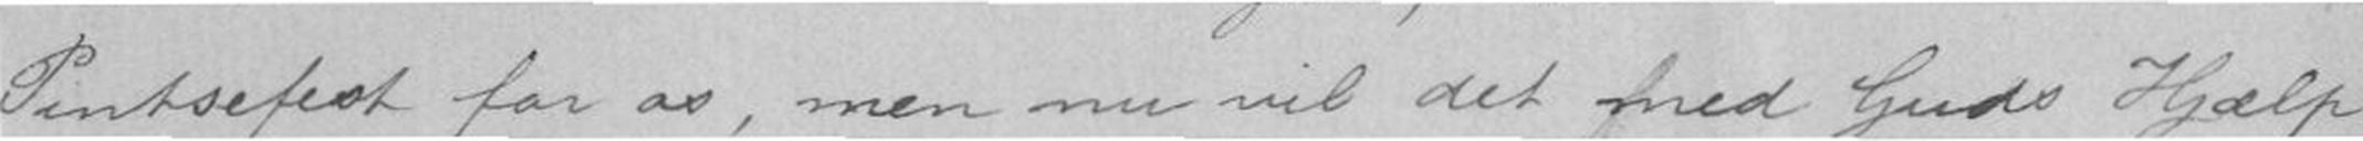Pintsefest for os, men nu vil det med Guds Hjælp
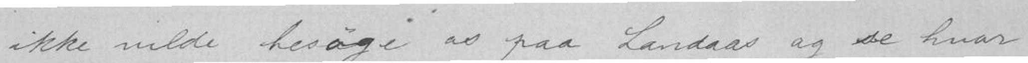ikke vilde besøge os paa Landaas og see hvor
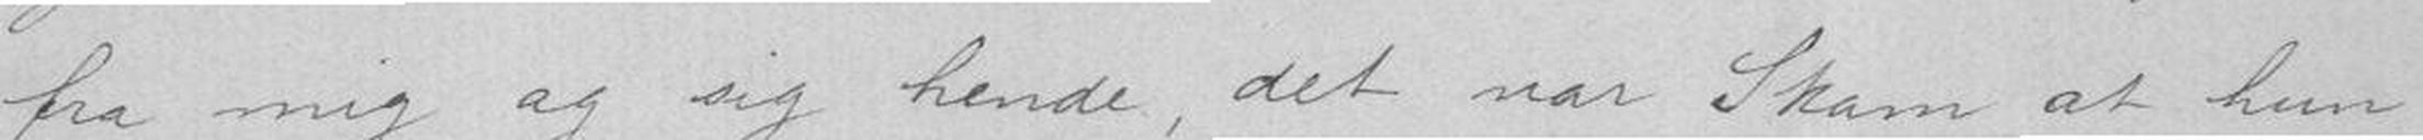fra mig og sig hende, det var Skam at hun
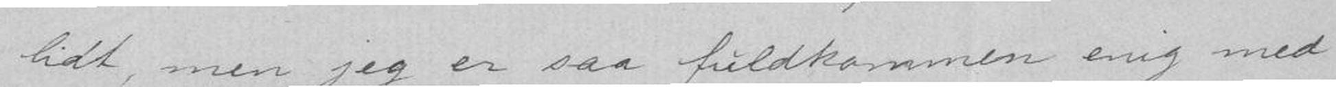lidt, men jeg er saa fuldkommen enig med
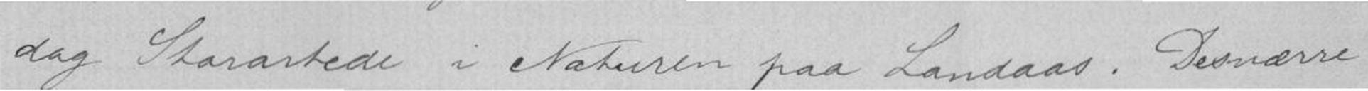dog Storartede i Naturen paa Landaas. Desværre
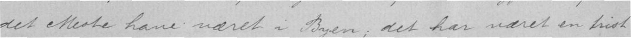det Meste have været i Byen; det har været en trist
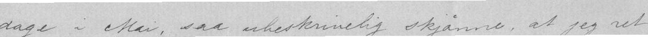dage i Mai, saa ubeskrivelig skjønne, at jeg ret
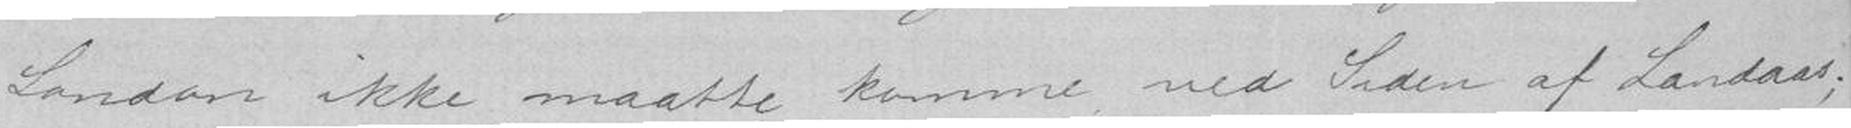London ikke maatte komme ved Siden af Landaas;
